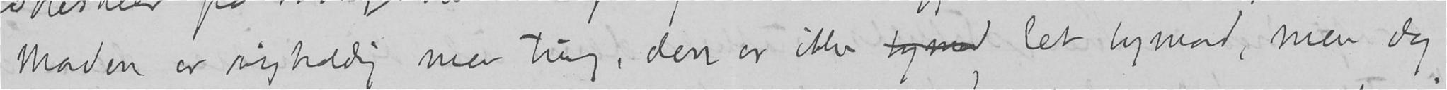Maden er righoldig men tung, den er ikke lymd let bymad, men dog
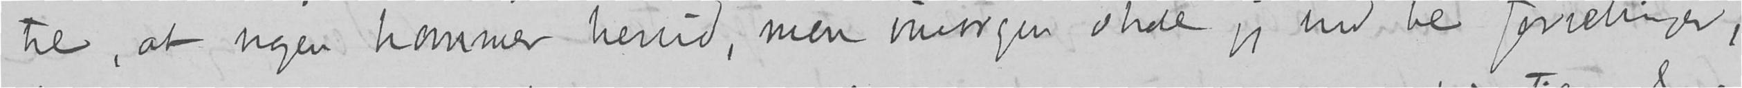til, at nogen kommer herud, men imorgen skal jeg ind til forretninger,
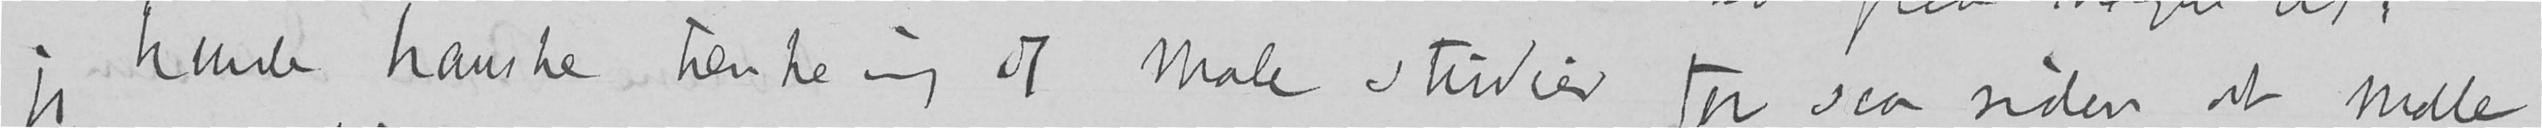jeg kunde kanske tænke mig at male studier for saa siden at male
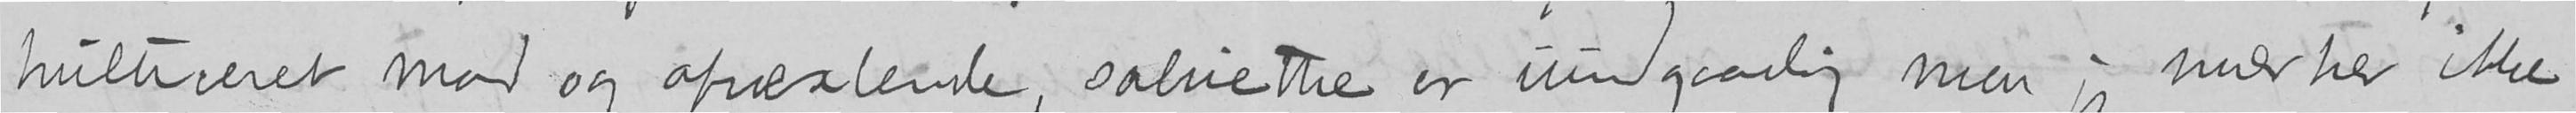kultiveret mad og afvæxlende, salviethe er uundgaaelig men jeg mærker ikke
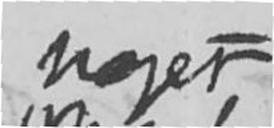noget
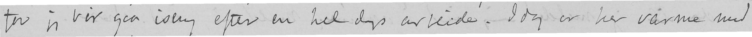for jeg bør gaa iseng efter en hel dags arbeide. Idag er her varme med
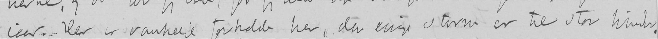iaar. Her er vankelige forholde her, den evige storm er til stor hinder,
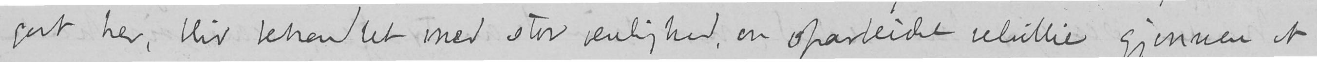godt her, blir behandlet med stor venlighed, en oparbeidet velvillie gjennem et
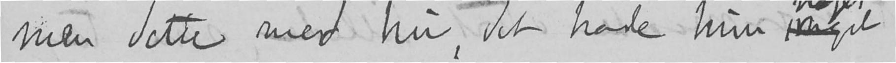men dette med hu, det havde hun meget
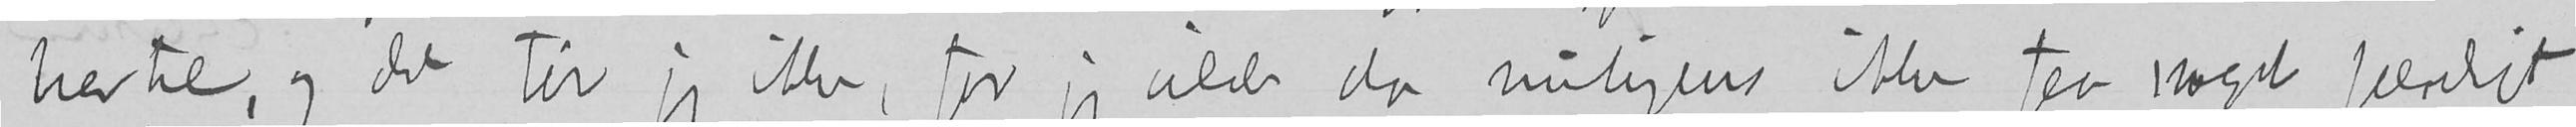hertil, og det tør jeg ikke, for jeg vilde da muligens ikke faa noget færdigt
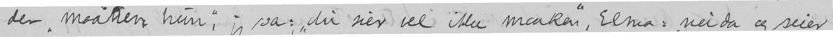der "maaken hun", jeg sa: "du sier vel ikke maaken", Elma: "neida eg seier
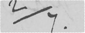2/7.
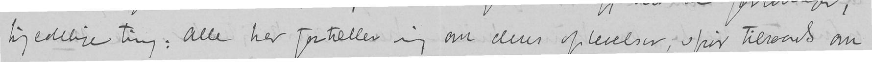kjedelige ting: Alle her fortæller mig om deres oplevelser, spør tilraads om
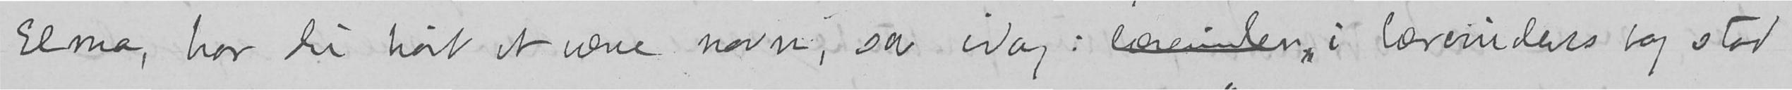Elma, har du hørt et værre navn, sa idag: lærerinden "i lærerindens bog stod
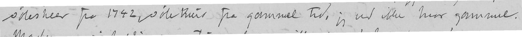sølvskeer fra 1742 sølvkrus fra gammel tid, jeg ved ikke hvor gammel.
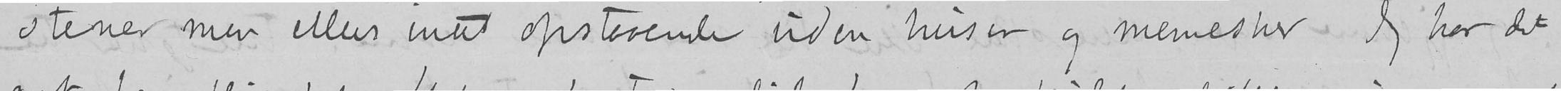stener men ellers intet opstaaende uden huser og mennesker. Jeg har det
